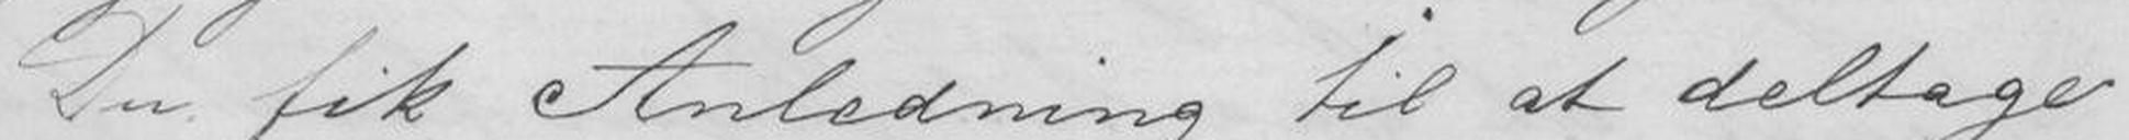Du fik Anledning til at deltage
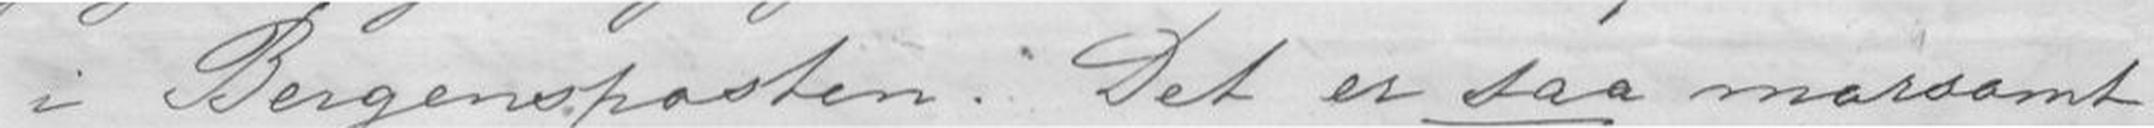i Bergensposten. Det er saa morsomt
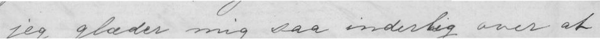jeg glæder mig saa inderlig over at
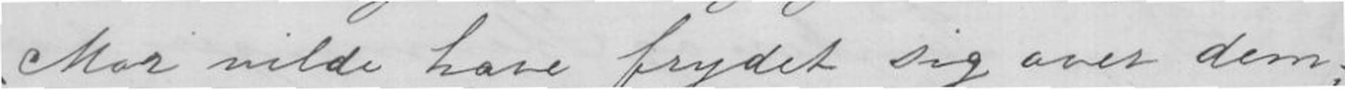Mor vilde have Frydet sig over dem;
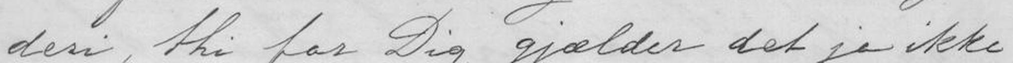deri, thi for Dig gjælder det jo ikke
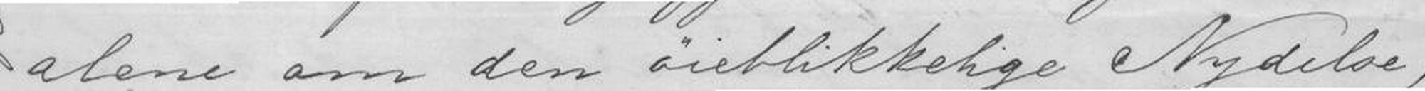alene om den øieblikkelige Nydelse,
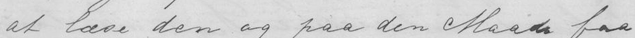at læse den og paa den Maade faa
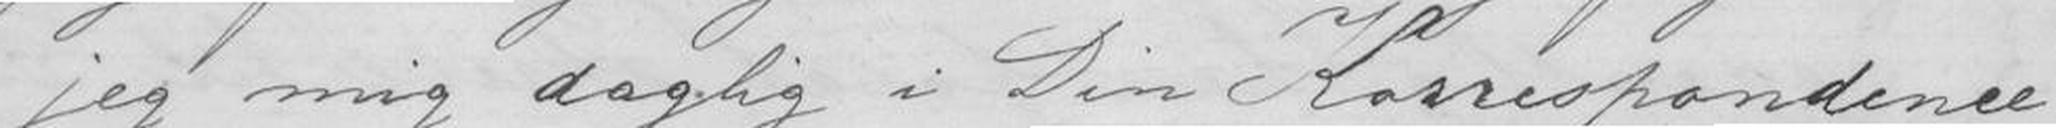jeg mig daglig i Din Korrespondance
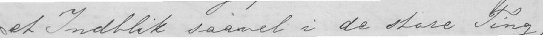et Indblik saavel i de store Ting,
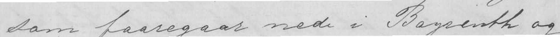som faaregaar nede i Bayrenth og
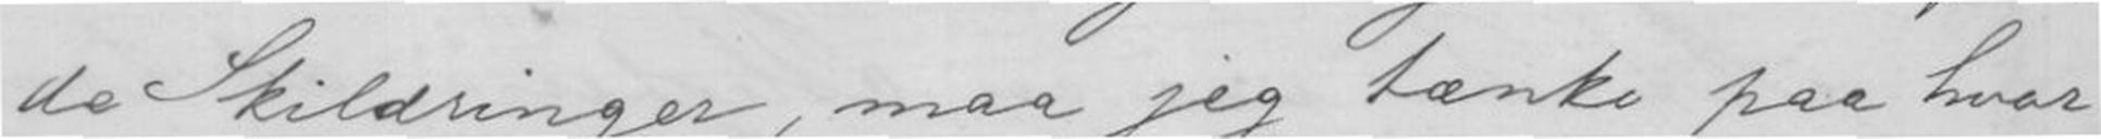de Skildringer, maa jeg tænke paa hvor
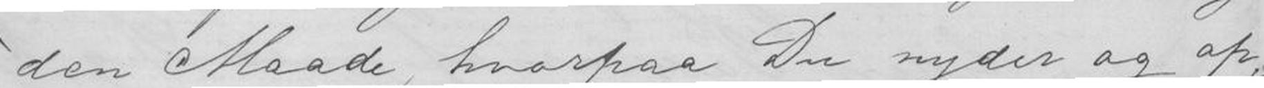den Maade hvorpaa Du nyder og op-
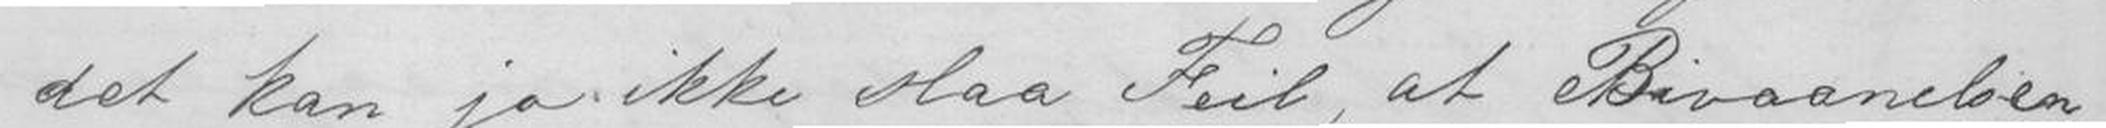det kan jo ikke slaa Feil, at Bivaanelsen
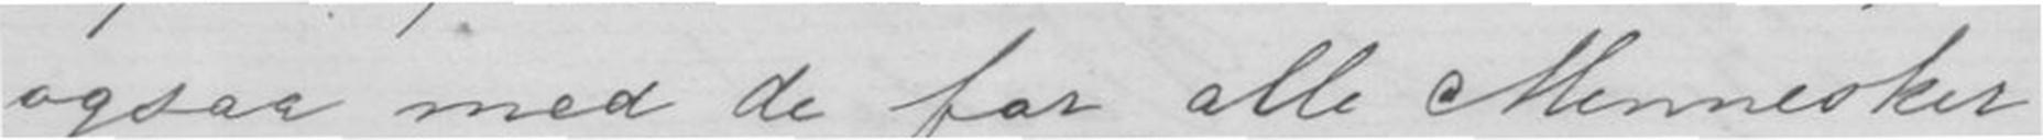ogsaa med de for alle Mennesker
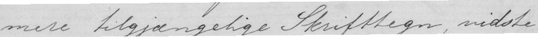mere tilgjeægelige Skriftegn, vidste
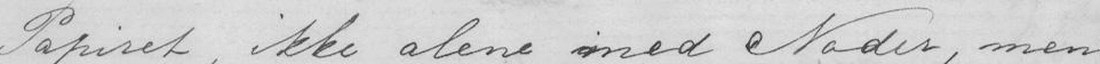Papiret, ikke alene med Noder, men
